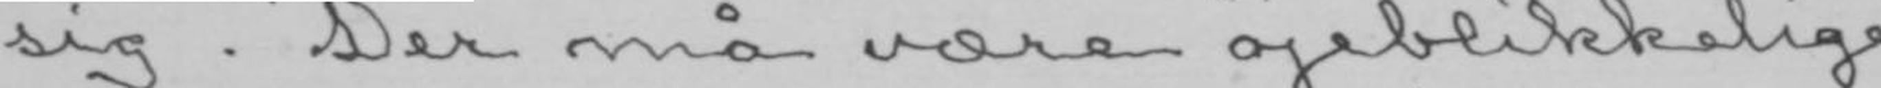sig. Der må være øjeblikkelige
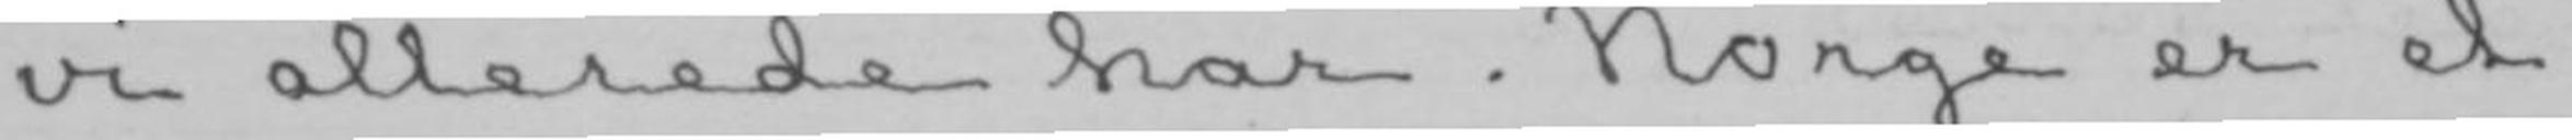vi allerede har. Norge er et
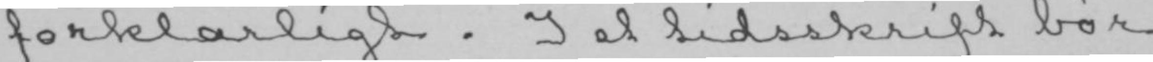forklarligt. I et tidsskrift bør
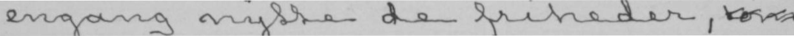engang nytte de friheder, som
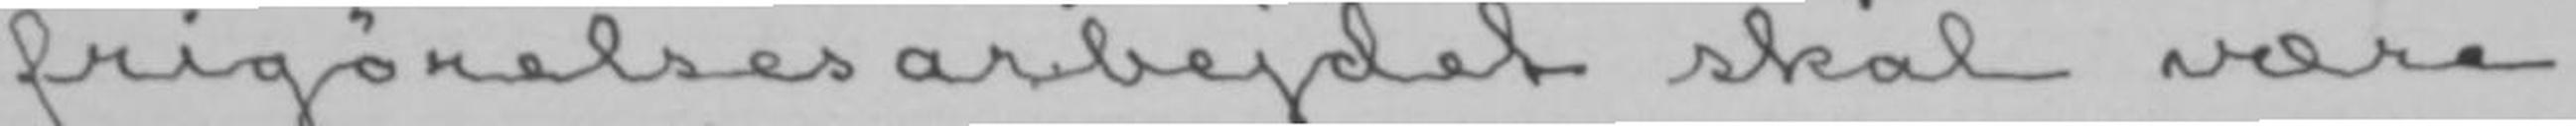frigørelsesarbejdet skal være
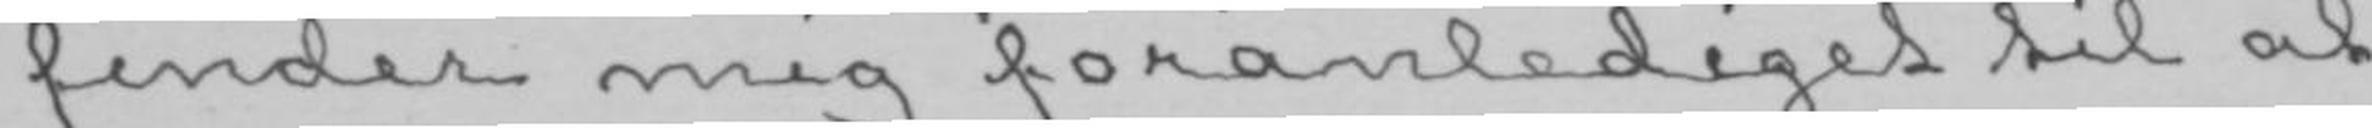finder mig foranlediget til at
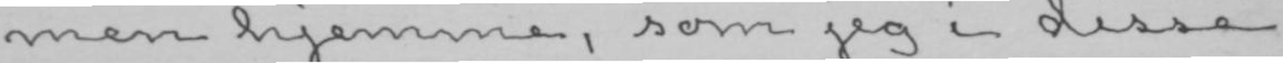men hjemme, som jeg i disse
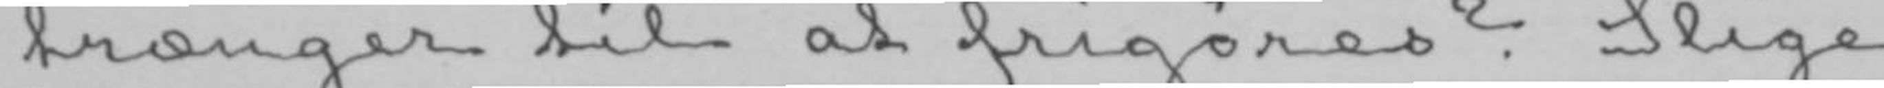trænger til at frigøres? Slige
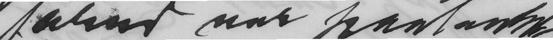forud var paatænkt
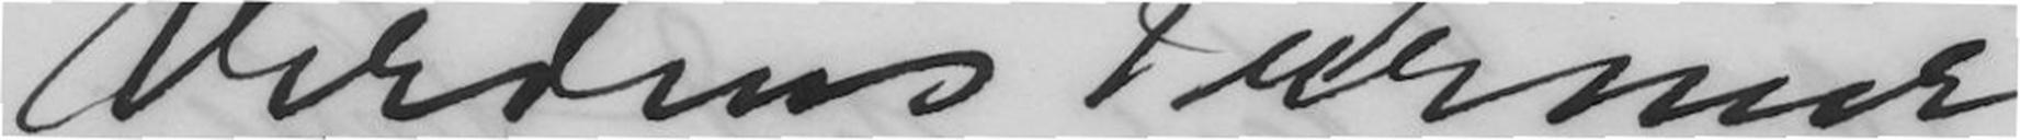Verdens Turnéer
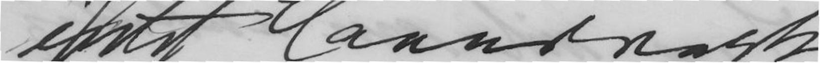intet Haandværk.
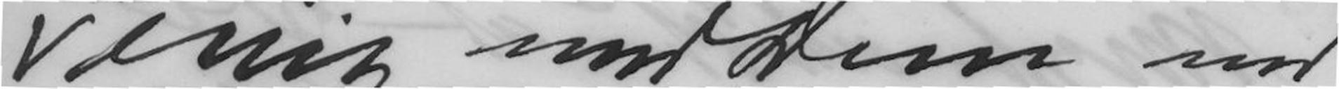enig med Dem end
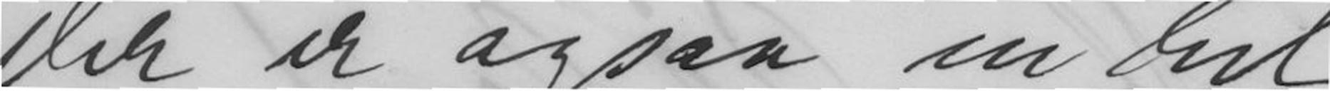der er ogsaa en hel
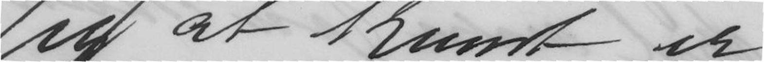jeg at Kunst er
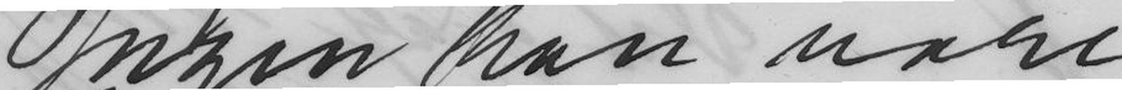Ingen kan være
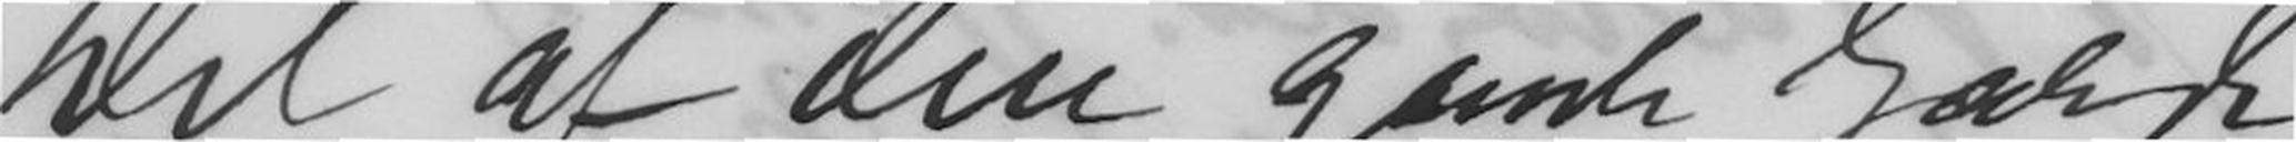Del af den gamle Garde.
